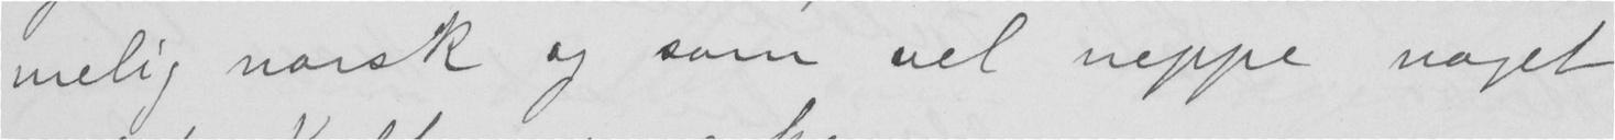melig norsk og som vel neppe noget
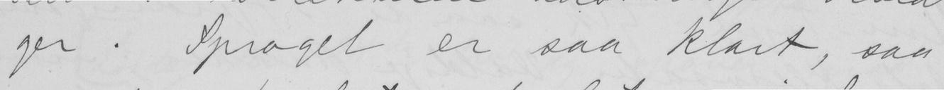ger. Sproget er saa klart, saa
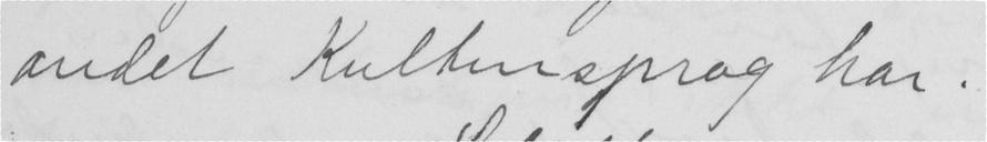andet Kultursprog har.
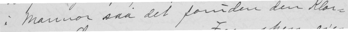i Marmor saa det foruden den Klar-
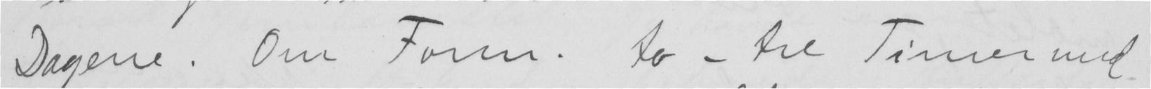Dagene. Om Form. to - tre Timer med
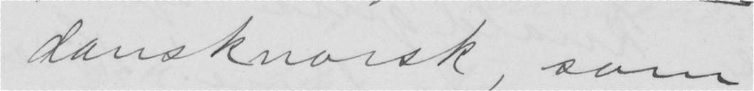dansknorsk, som
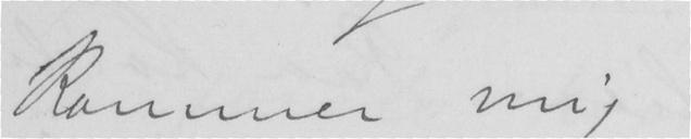kommer mig
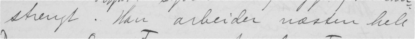strengt. Han arbeider næsten hele
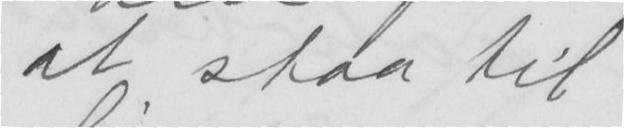at staa til
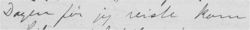Dagen før jeg reiste kom
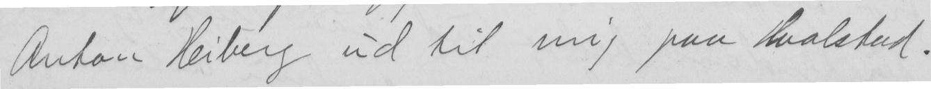Anton Heiberg ud til mig paa Hvalstad.
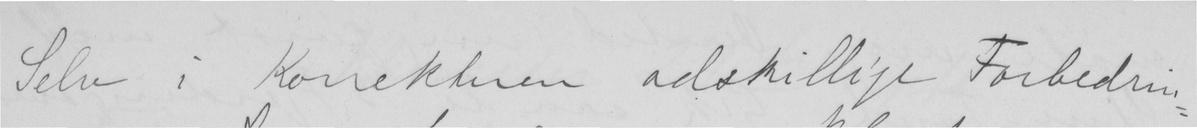Selv i Korekturen adskillige Forbedrin-
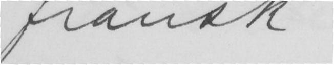fransk
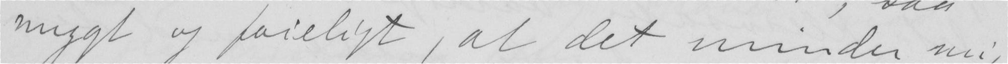mygt og føieligt, at det minder mig
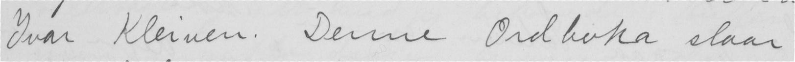Ivar Kleiven. Denne Ordboka slaar
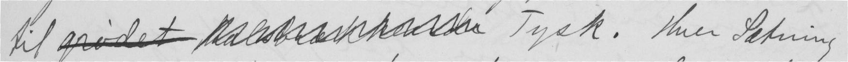til  Tysk. Hver Sætning
